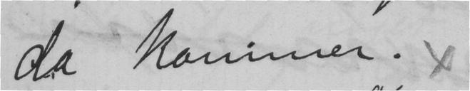da kommer. x
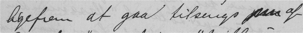ligefrem at gaa tilsengs  af
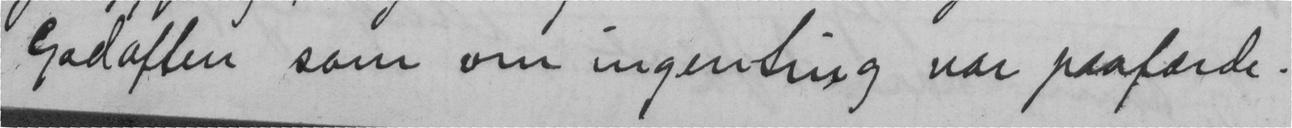Godaften som om ingenling var paafærde.
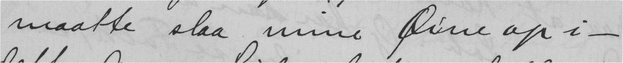maatte slaa mine Øine op i
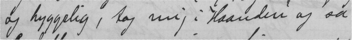og hyggelig, tog mig i Haanden og sa
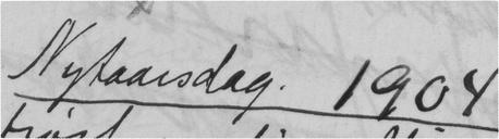Nytaarsdag. 1904
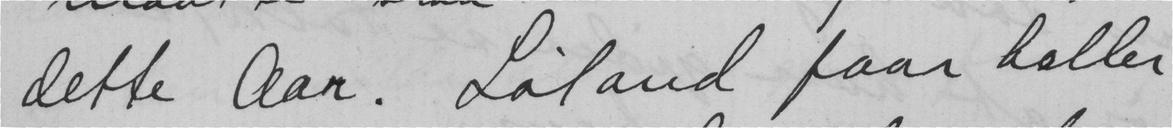dette aar. Løland faar heller
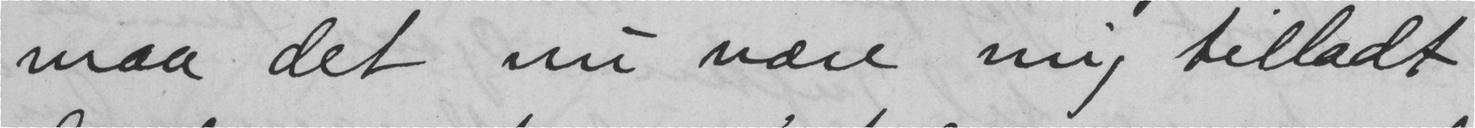maa det nu være mig tilladt
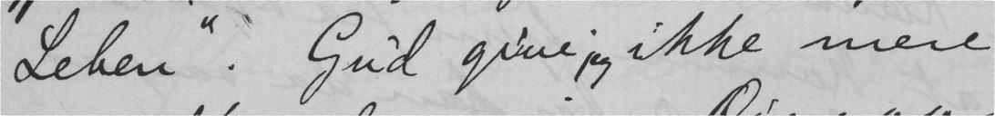Leben". Gud give jeg ikke mere
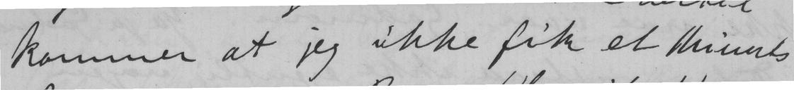kommer at jeg ikke fik et minuts
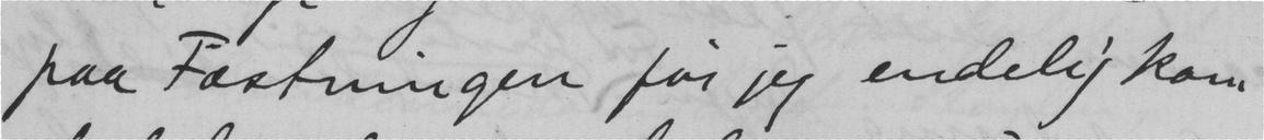paa Fæstningen før jeg endelig kom
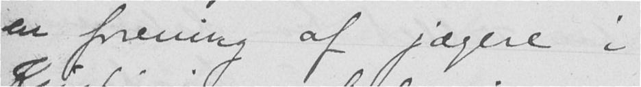en forening af jægere i
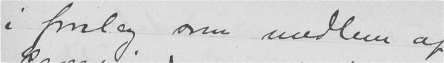i forslag som medlem af
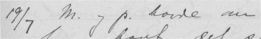19/7 M. og p. havde om
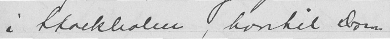i Stockholm, hvortil Don
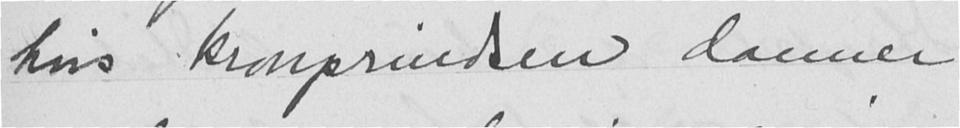hvis kronprindsen danner
